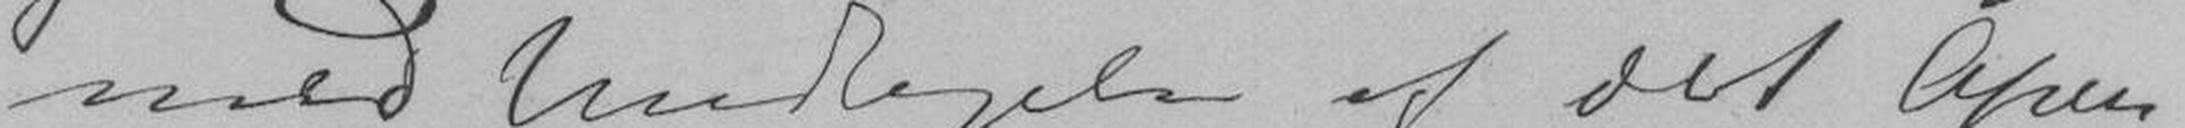med Undtagelse af det Afven
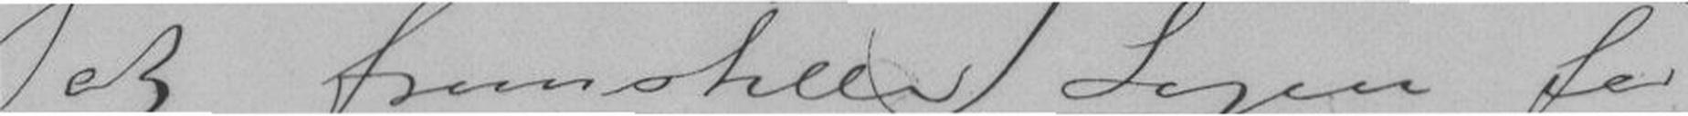at fremstille Sagen for
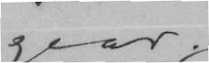gaar. -
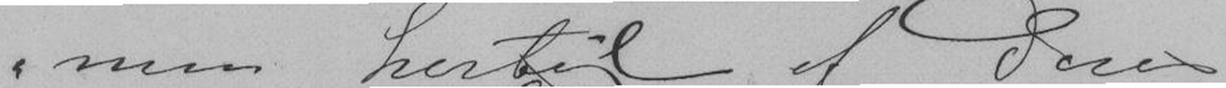men hertil af Deres
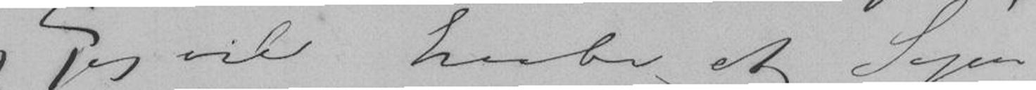og jeg vil haabe at Sagen
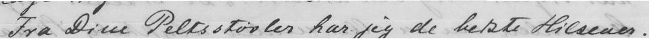Fra Dine Peltsstøvler har jeg de bedste Hilsener.
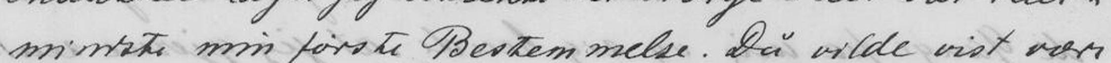mindste min første Bestemmelse. Du vilde vist, være
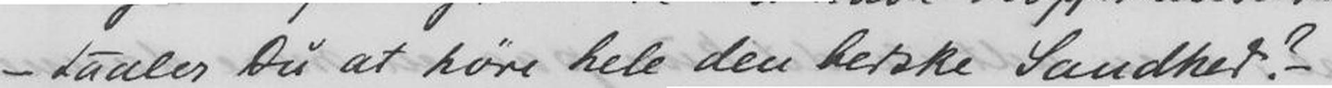- taaler Du at høre hele den bedske Sandhed?
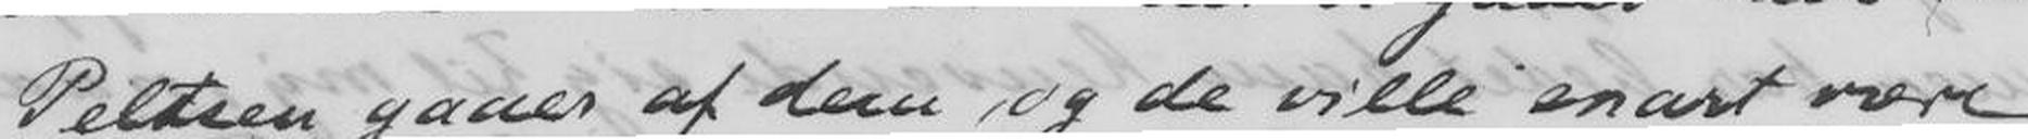Peltsen gaaer af dem og de ville snart være
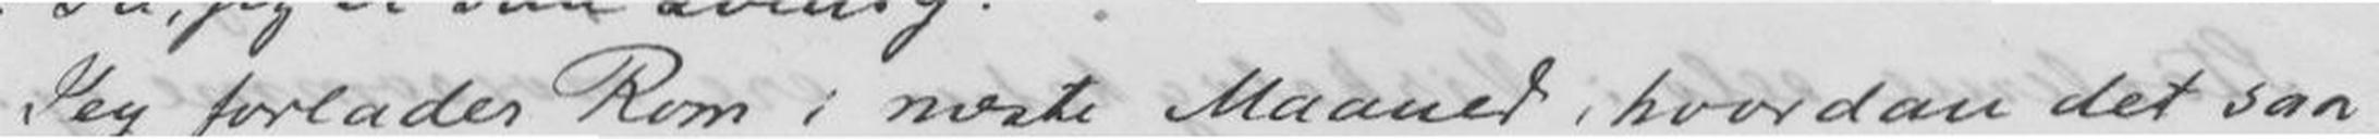Jeg forlader Rom i næste Maaned, hvordan det saa
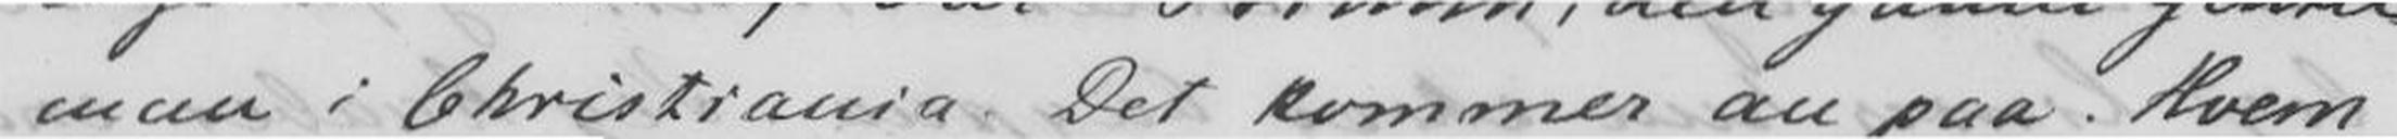gamle Gentleman i Christiania. Det kommer an paa.
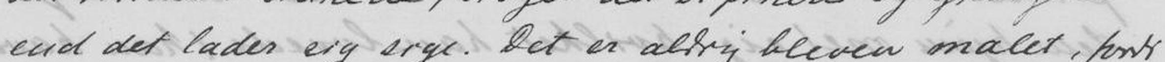end det lader sig sige. Det er aldrig bleven malet, fordi
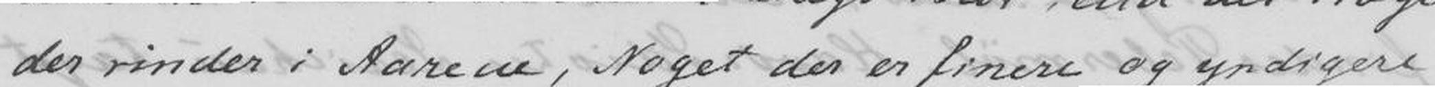der rinder i Aarene, Noget der er finere og yndigere
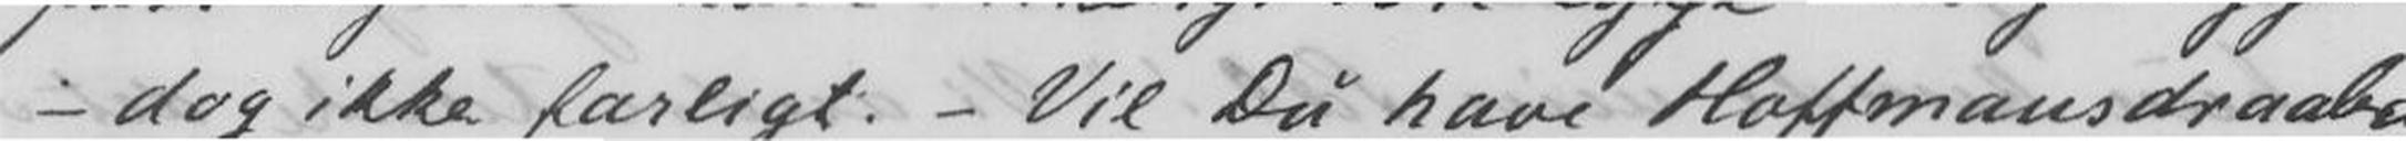- dog ikke farligt. - Vil Du have Hoffmansdraaber
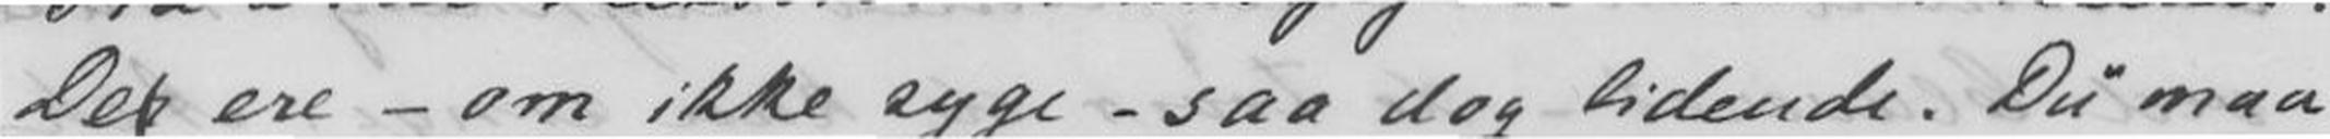De ere - om ikke syge - saa dog lidende. Du maa
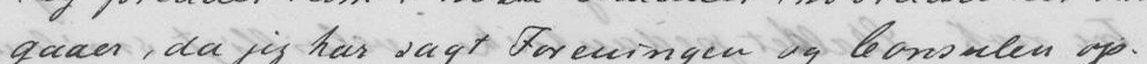gaaer, da jeg har sagt Foreningen og Consulen op.
